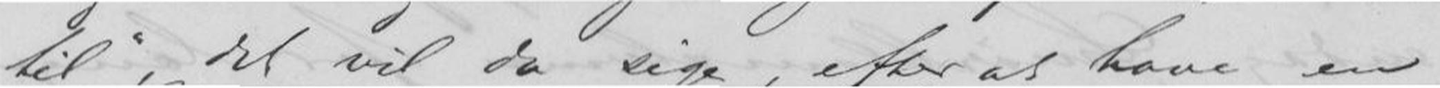til", det vil da sige, efter at have en
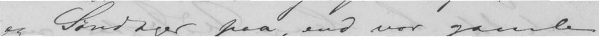og Søndager paa, end vor gamle
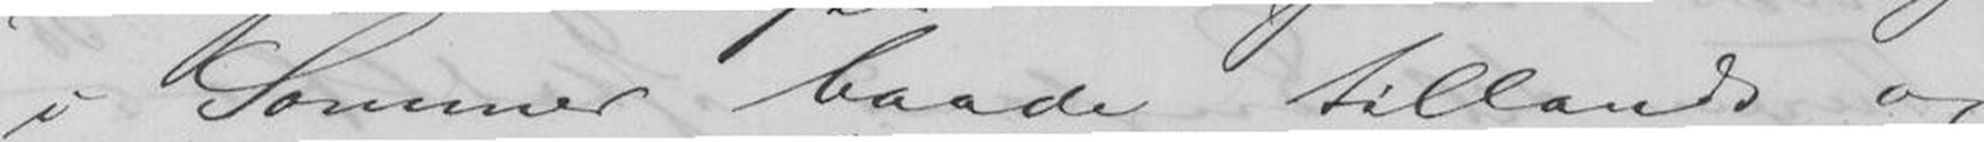i Sommer baade tillands og
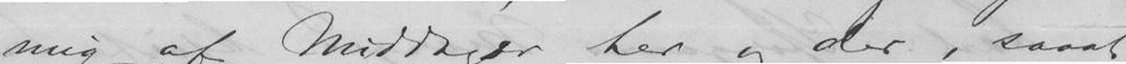mig af Middager her og der, saaat
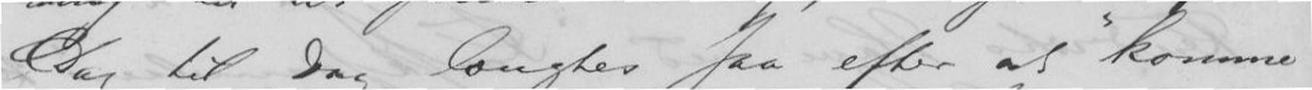Dag til Dag længtes saa efter at "komme
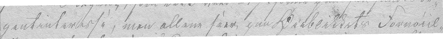gentinteresse, men allene seer paa Øieblikkets Fornøiel-
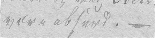være absurd. –
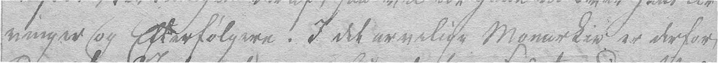vinger og Efterfølgere. I det arvelige Monarkie er derfor
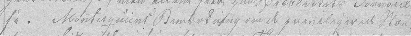se. Montesquieus Bemærkning om de priviligerede Stæn-
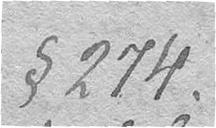§ 274.
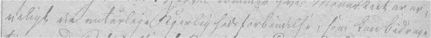veligt den naturlige Kjærlighedsforbindelse, som kan bidrage
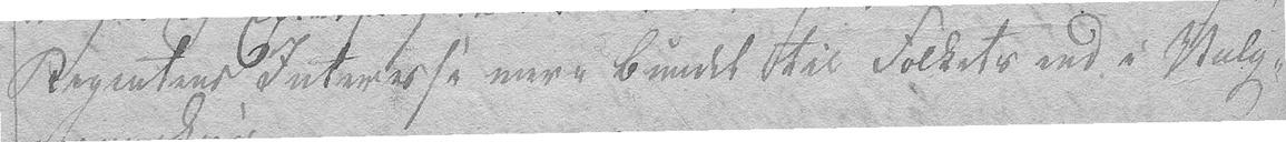Regentens Interesse mere bundet til Folkets end i Valg-
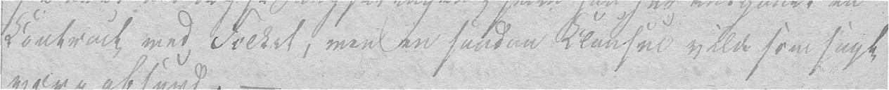Contract med Folket, men en saadan Clausul vilde som sagt
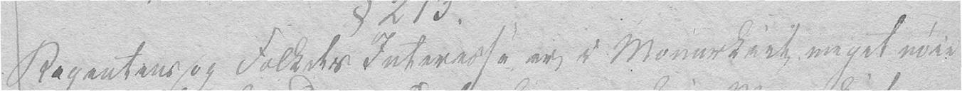Regentens og Folkets Interesse er i Monarkiet meget nøie
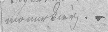monarkier. –
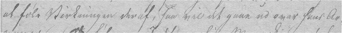at føle Virkningen deraf, saa vil det gaae ud over hans Ar-
